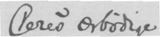Deres ærbødige
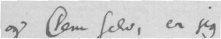og Dem selv, er jeg
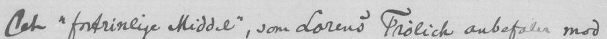Det "fortrinlige Middel", som Lorens Frølich anbefaler mod
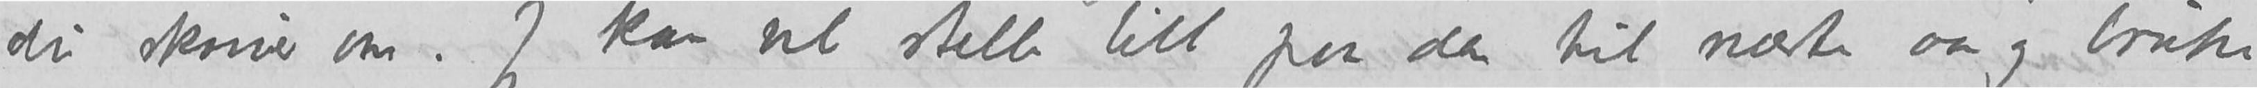du skriver om. Jeg kan vel stelle litt paa den til næste aar og bruke
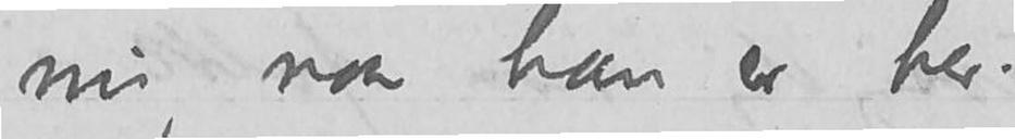nu, naar han er her
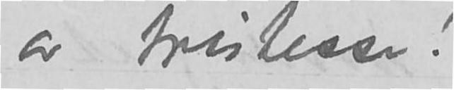av tristesse!
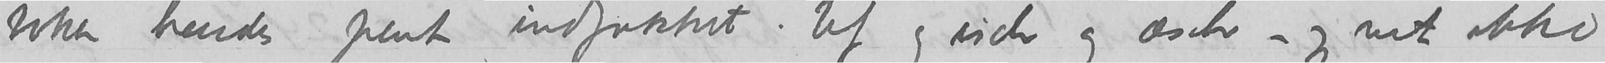boka hendes pent indpakket. Uf og isch og æsch – jeg vet ikke
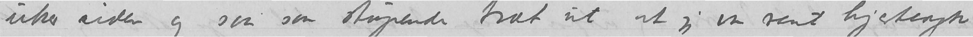uker siden og saa saa stupende træt ut at jeg var rent hjertesyk
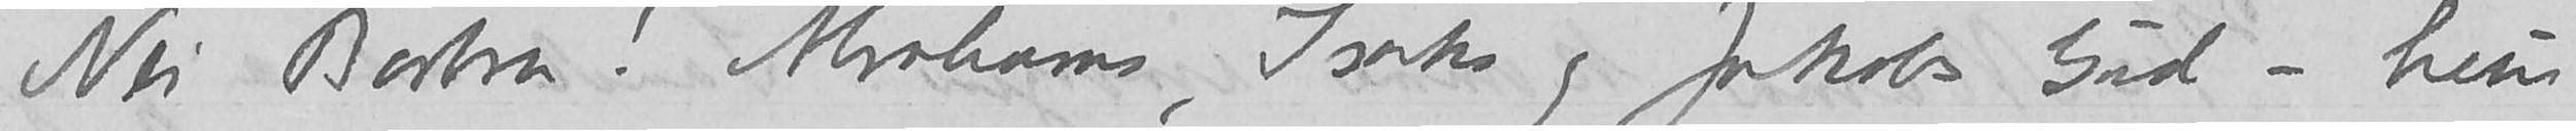Nei Barbra! Abrahams, Isaks og Jakobs Gud – hun
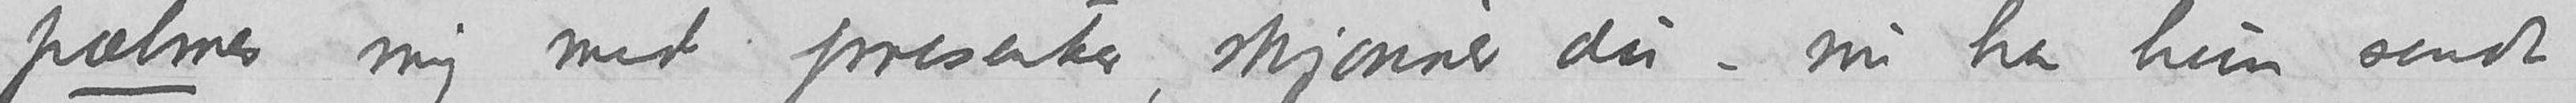pælmer mig med presenter skjønner du – nu har hun sendt
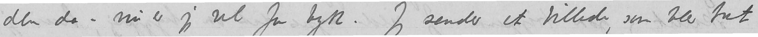den da – nu er jeg vel for tyk. Jeg sender et billede, som blev tat
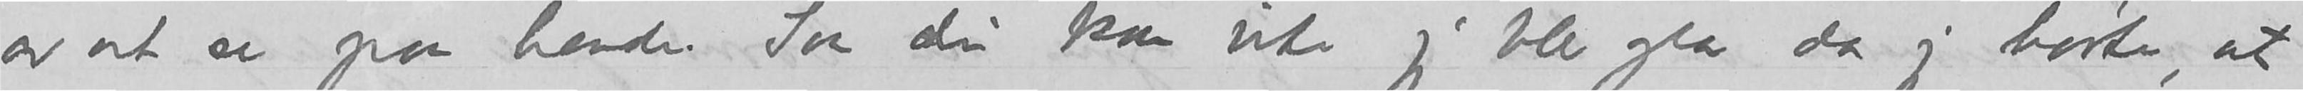av at se paa hende. Saa du kan vite jeg blev gla da jeg hørte, at
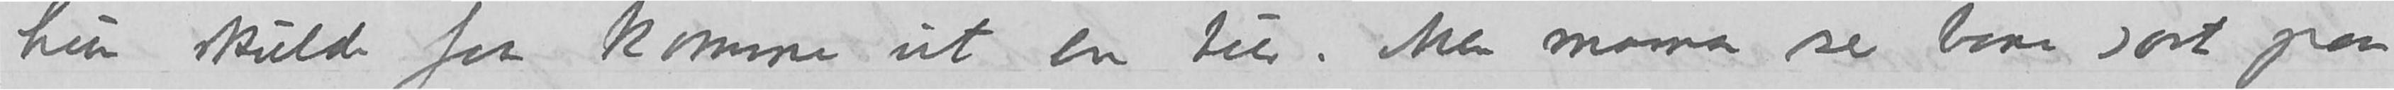hun skulde faa komme ut en tur. Men mama ser bare sort paa
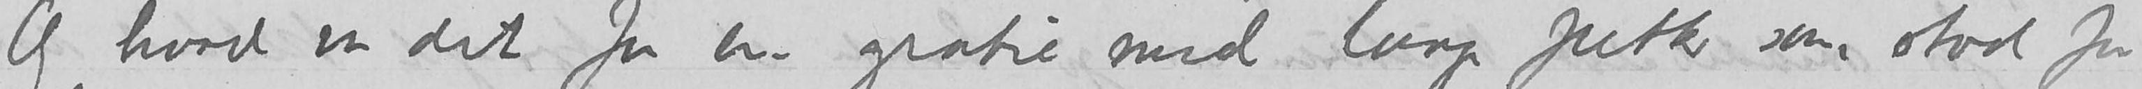Og hvad er det for en gratie med lange fletter som stod for
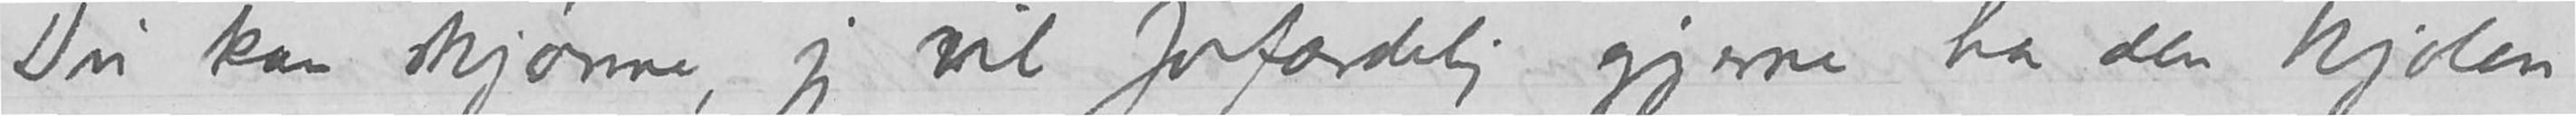Du kan skjønne, jeg vil forfærdelig gjerne ha den kjolen
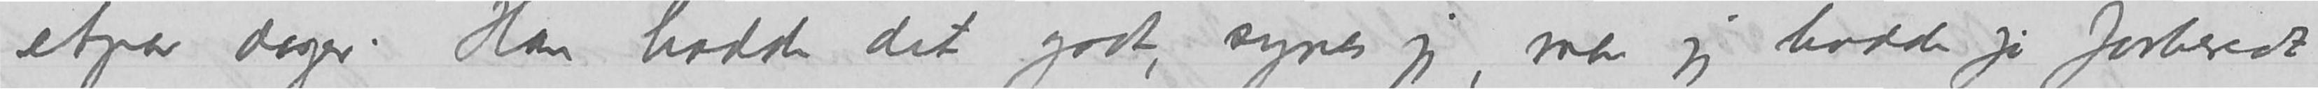etpar dager. Han hadde det godt, synes jeg, men jeg hadde jo forberedt
